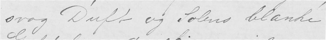svag Duft og Solens blanke
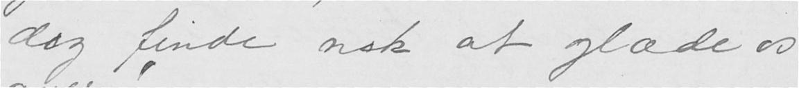dog finde nok at glæde os
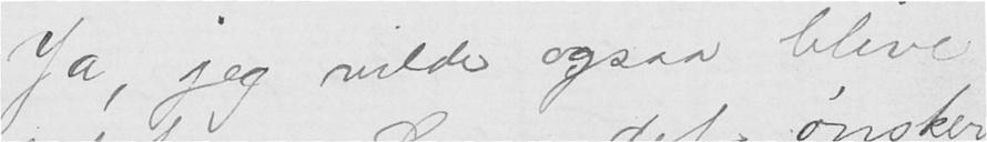Ja, jeg vilde ogsaa blive
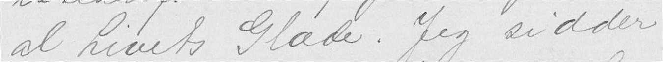al Livets Glæde. Jeg sidder
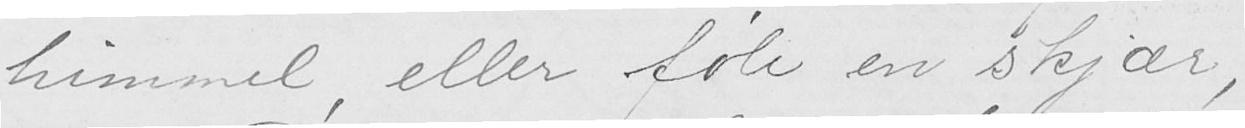himmel, eller føle en skjær,
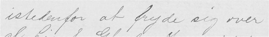istedenfor at fryde sig over
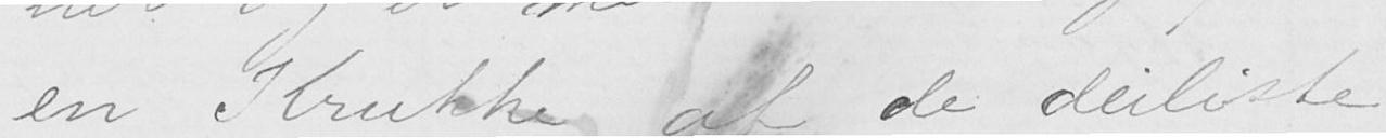en Krukke af de deiligste
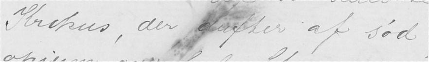Krokus, der dufter af sød
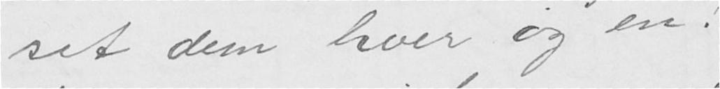set dem hver og en!
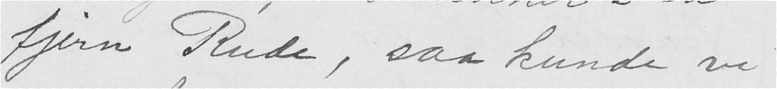fjern Rude, saa kunde vi
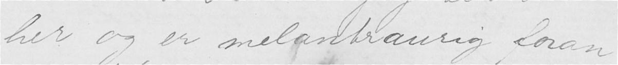her og er melantraurig foran
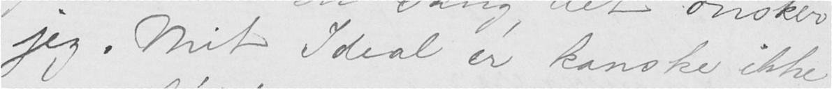jeg. Mit Ideal er kanske ikke
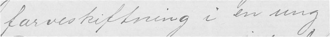farveskiftning i en ung
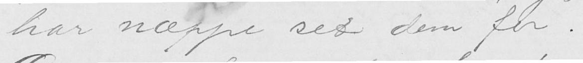har næppe set dem før.
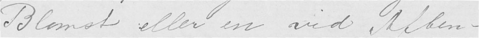Blomst eller en vid Aften-
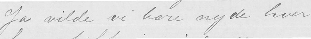Ja vilde vi bare nyde hver
